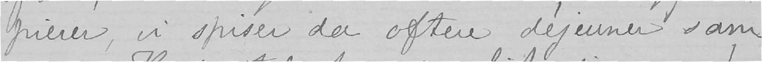pierer, vi spiser da oftere dejeuner sam-
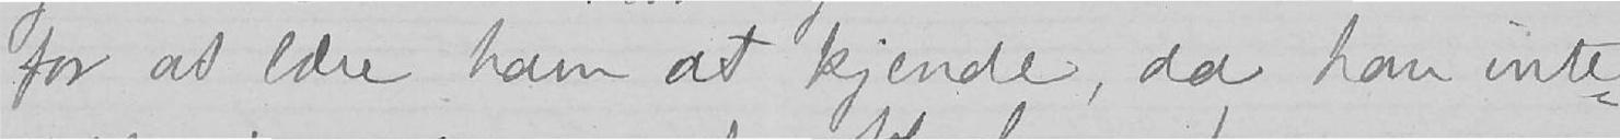for at lære ham at kjende, da han inte-
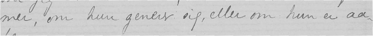mer, om hun generer sig, eller om hun er aa-
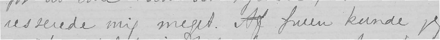resserede mig meget. Af fruen kunde jeg
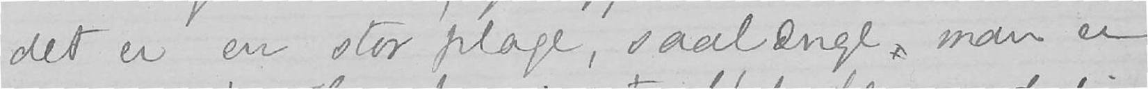det er en stor plage, saalænge, man er
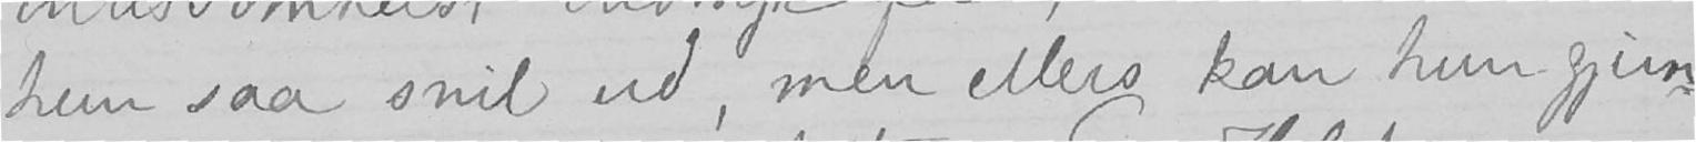hun saa snil ud, men ellers kan hun gjem-
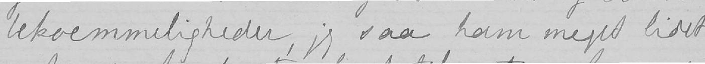bekvemmeligheder, jeg saa ham meget lidet
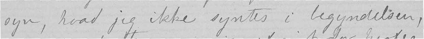syn, hvad jeg ikke syntes i begyndelsen,
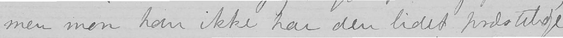men mon han ikke har den lidet præstelige
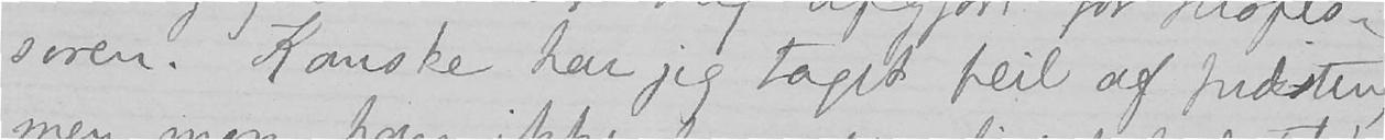soren. Kanske har jeg taget feil af præsten,
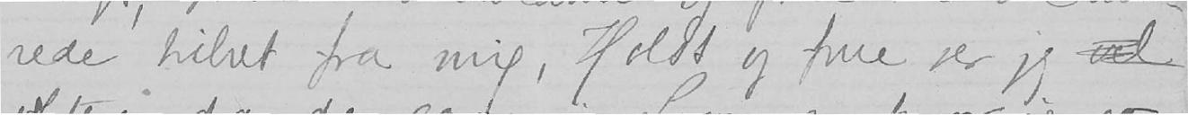rede hilset fra mig, Holst og frue ser jeg vel
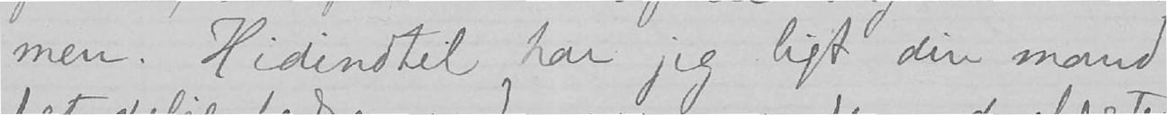men. Hidindtil har jeg ligt din mand
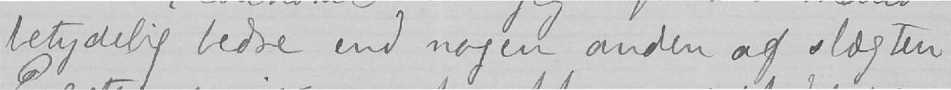betydelig bedre end nogen anden af slægten.
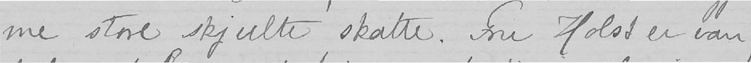me store skjulte skatte. Fru Holst er van-
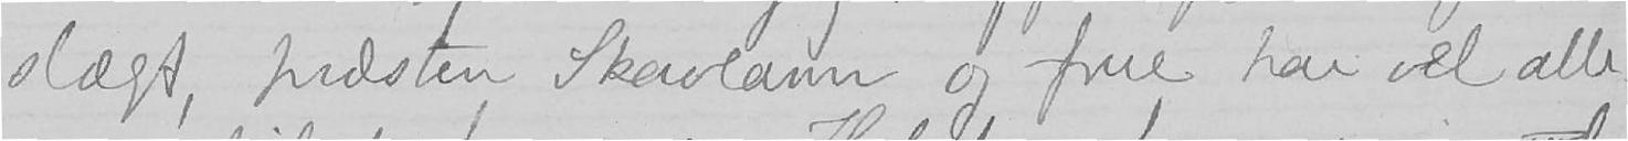slægt, præsten Skavlann og frue har vel alle-
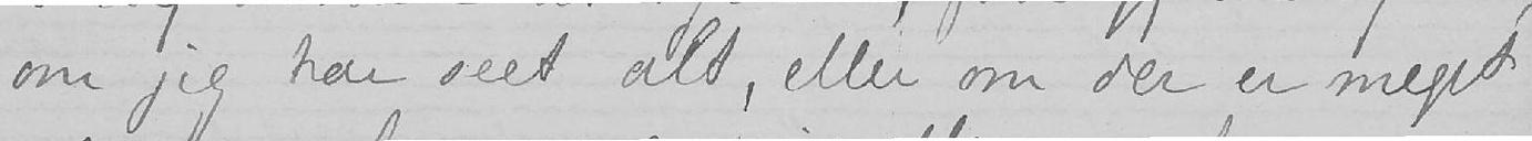om jeg har seet alt, eller om der er meget
